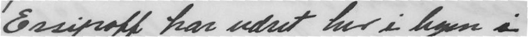Essipoff har været her i byen i
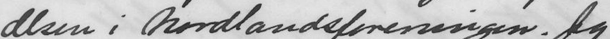Olsen i Nordlandsforeningen. Jeg
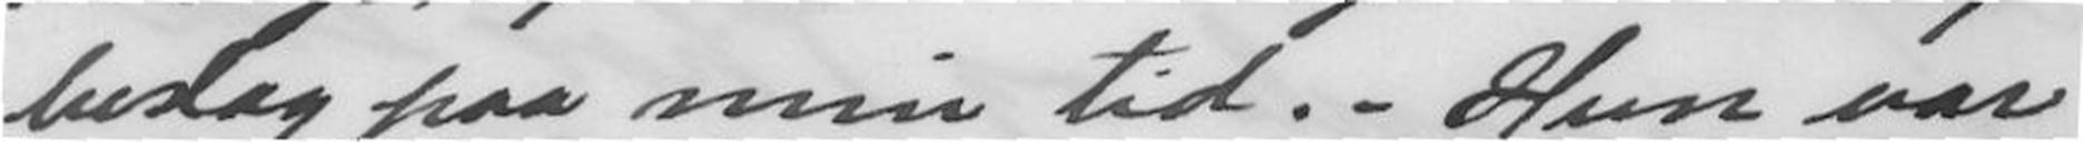beslag paa min tid. - Hun var
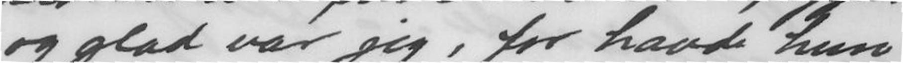og glad var jeg, for haavde hun
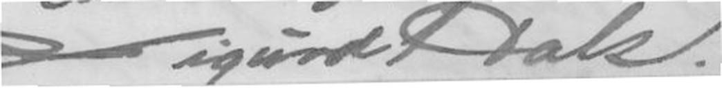Sigurd Hals.
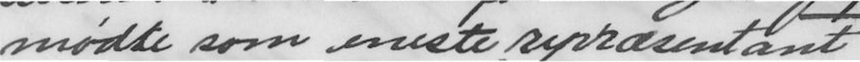mødte som eneste repræsentant
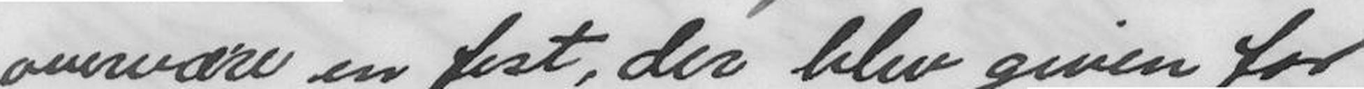overvære en fest, der blev given for
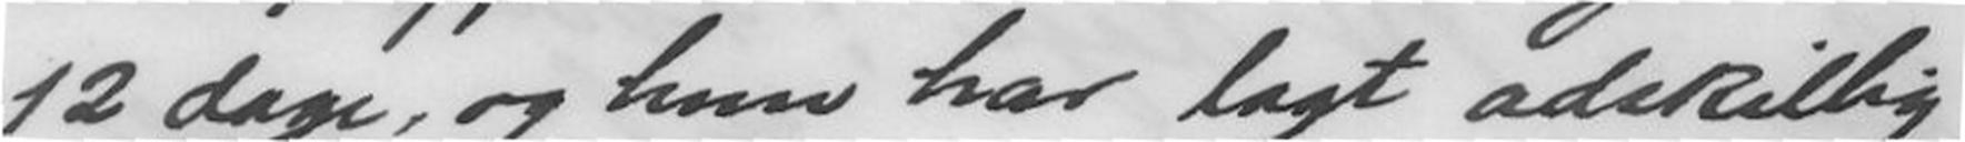12 dage, og hun har lagt adskillig
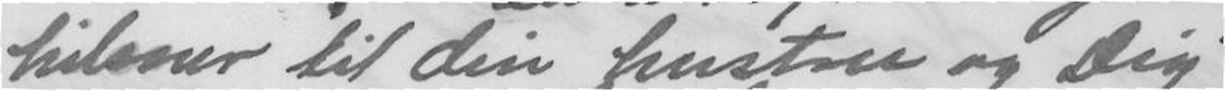hilsener til din hustru og Dig
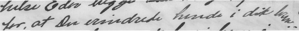for, at Du erindrede hende i dit brev.
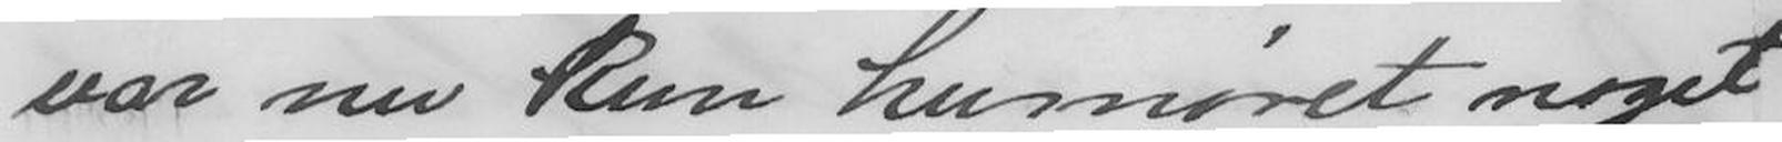var nu kun humøret noget
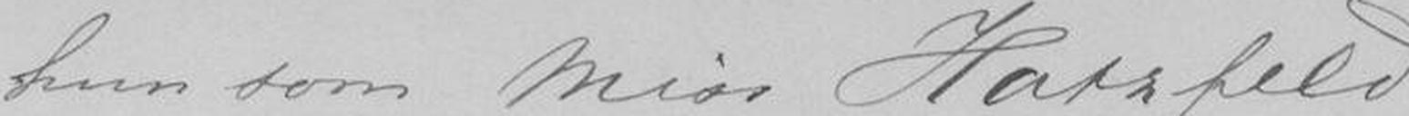hun som Miss Hatsfeld
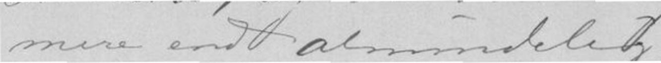mere end almindelig
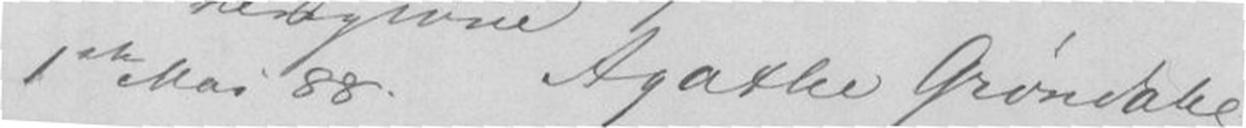1ste Mai 88. Agathe Grøndahl
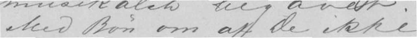Med Bøn om at De ikke
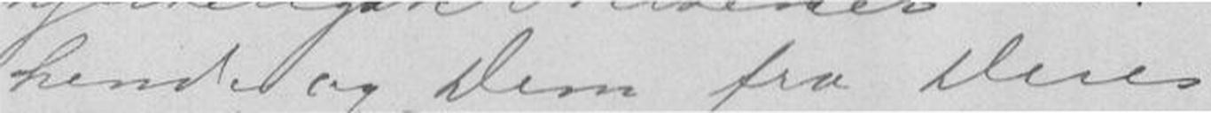hende og Dem fra Deres
